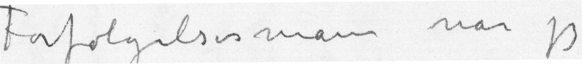Forfølgelsesmani når jeg
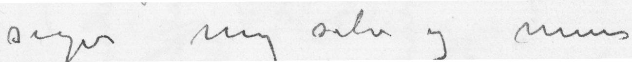siger mig selv og mine
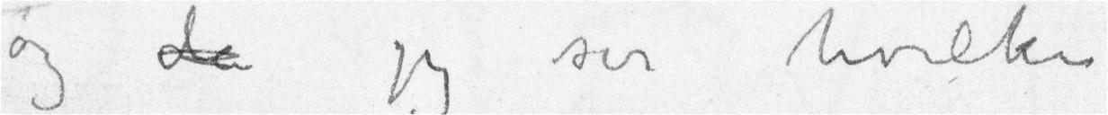og da jeg ser hvilke
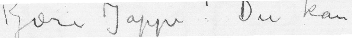Kjære Jappe! Du kan
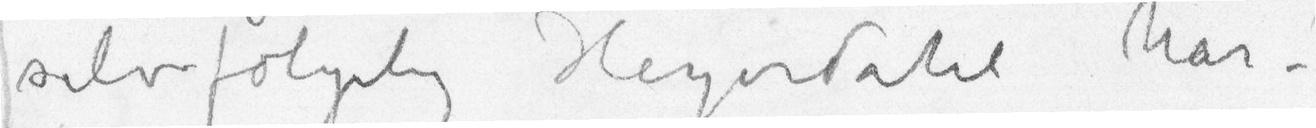selvfølgelig Heyerdahl har –
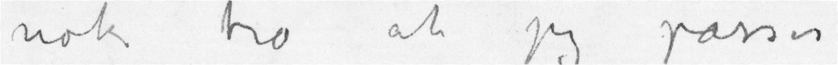nok tro at jeg passer
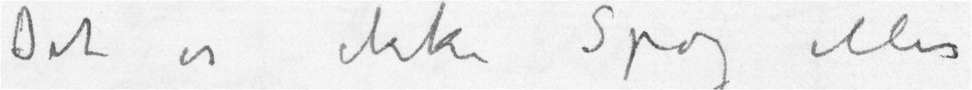Det er ikke Spøg eller
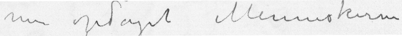mer opdaget Menneskerne
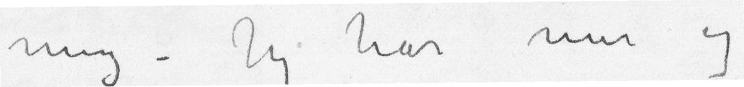mig – Jeg har mer og
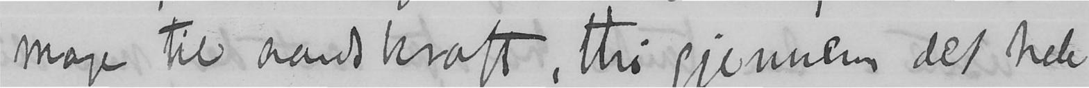mage til aandskraft, thi gjemmem det hele
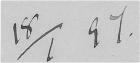18/1 97
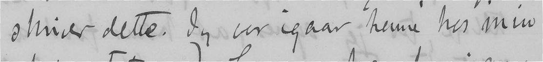skriver dette. Jeg var igaar henne hos min
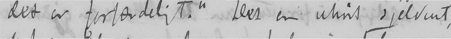det er forfærdeligt." Det en uhørt sjeldent,
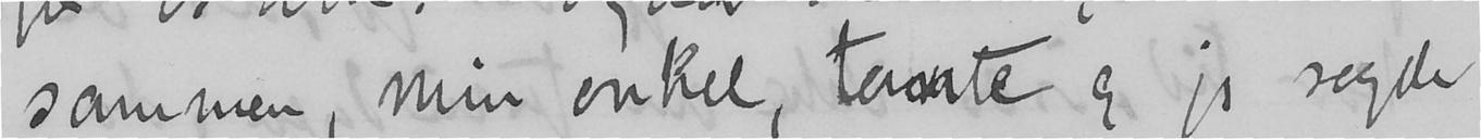sammen, min onkel, tante og jeg sagde
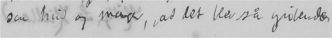san hvid og mager, at det blev så gribende
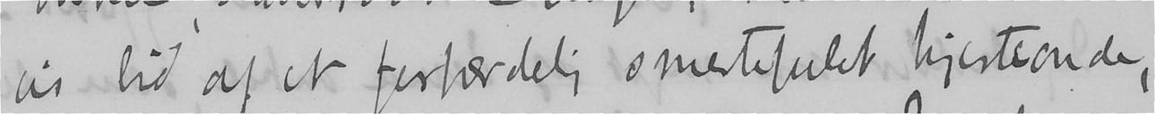vis lid af et forfærdelig smertefuldt hjerteonde,
